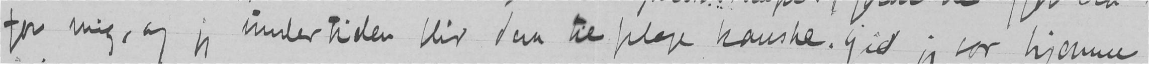for mig, og jeg undertiden blir dem til plage kanske. Gid jeg var hjemme
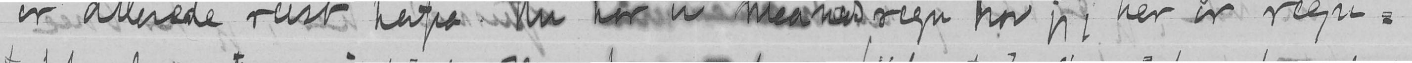er allerede reist herfra. Nu har vi maanedsregn tror jeg, her er regn-
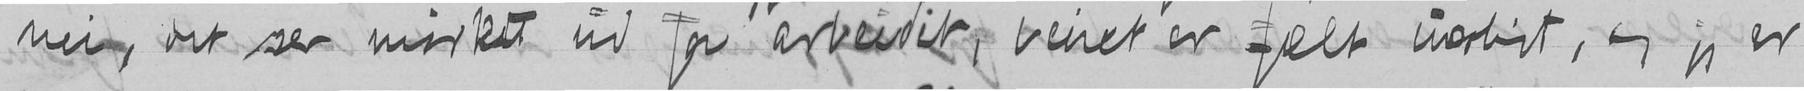nei, det ser mørkt ud for arbeidet, veiret er fælt uroeligt, og jeg er
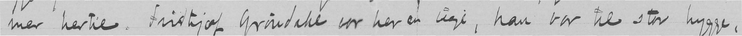mer hertil. Fridtjof Grøndahl var her en uge, han var til stor hygge,
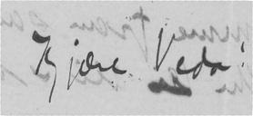Kjære Veda!
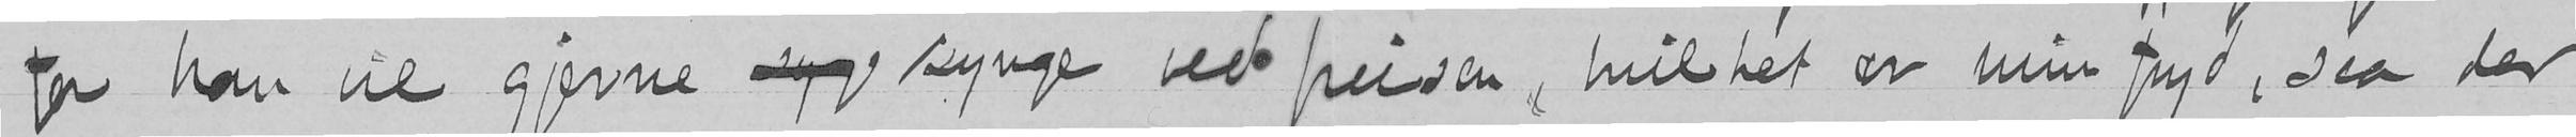for han vil gjerne syge synge ved peisen, hvilket er min fryd, saa der
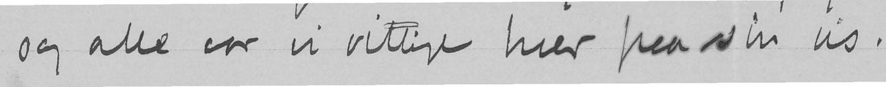og alle var vi vittige hver paa sin vis.
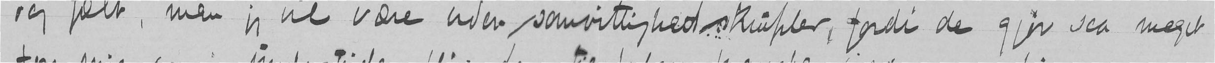og fælt, men jeg vil være uden samvittighedskrupler, fordi de gjør saa meget
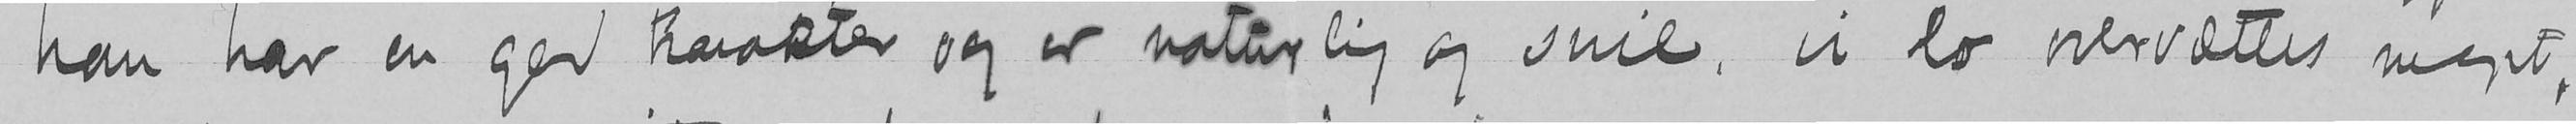han har en god karakter og er naturlig og snil, vi lo overvættes meget,
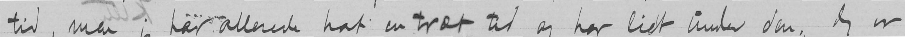tid, men jeg har allerede hat en træt tid og har lidt under den. Jeg er
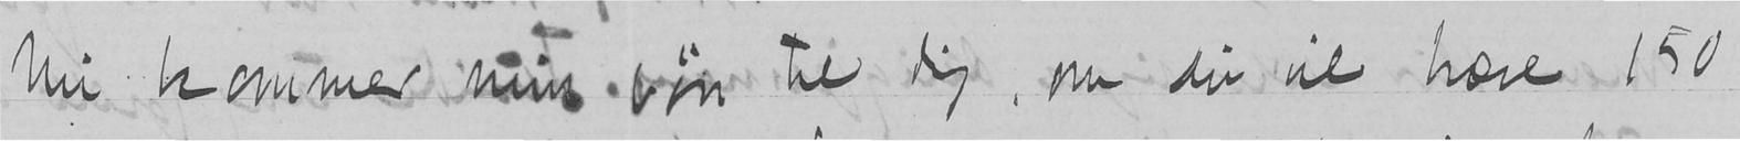Nu kommer min bøn til dig, om du vil hæve 150
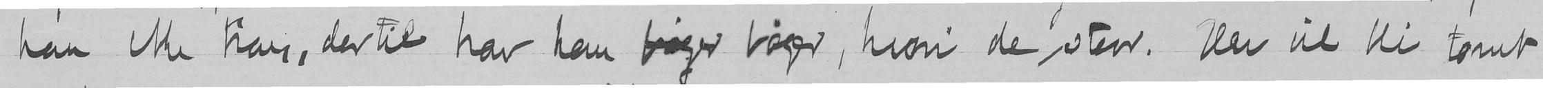han ikke kan, dertil har han bager bøger, hvori de staar. Her vil bli tomt
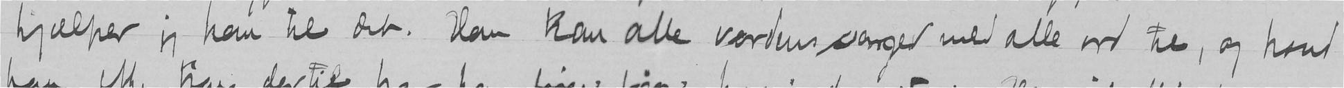hjælper jeg ham til det. Han kan alle verdens sanger med alle ord til, og hvad
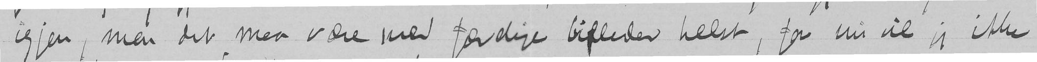igjen, men det maa være med færdige billeder helst, for nu vil jeg ikke
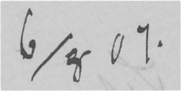6/8 07.
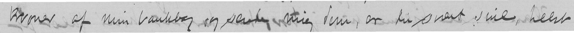kroner, af min bankbog og sende mig dem, er du svært snil, helst
